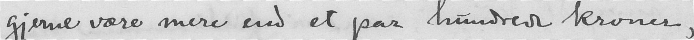gjerne være mere end et par hundrede kroner,
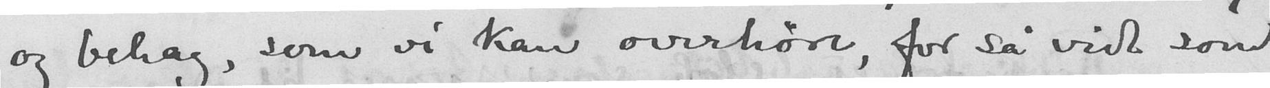og behag, som vi kan overhøre, for så vidt som
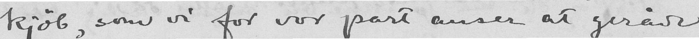kjøb, som vi for vor part anser at geråde
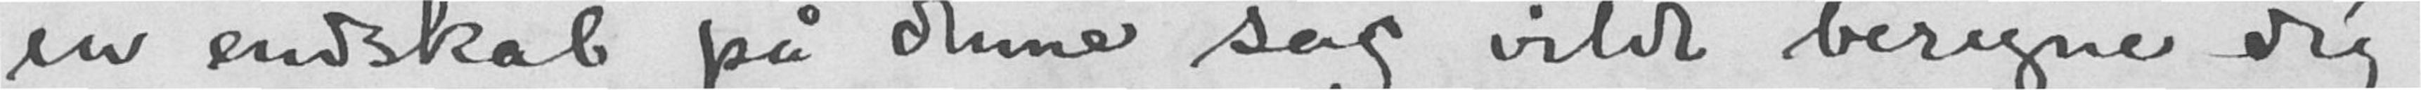en endskab på denne sag vilde beregne dig
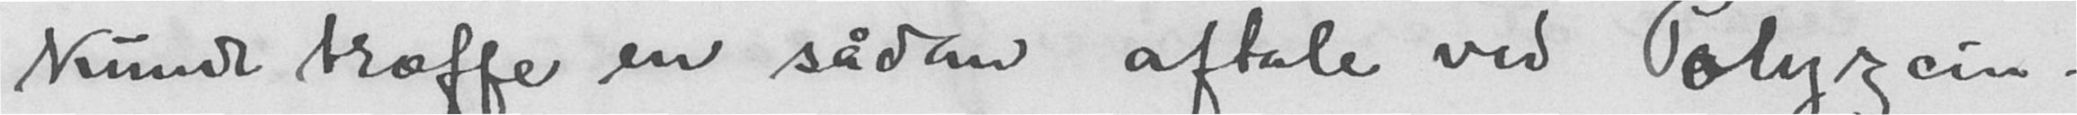kunde træffe en sådan aftale ved Polyzein-
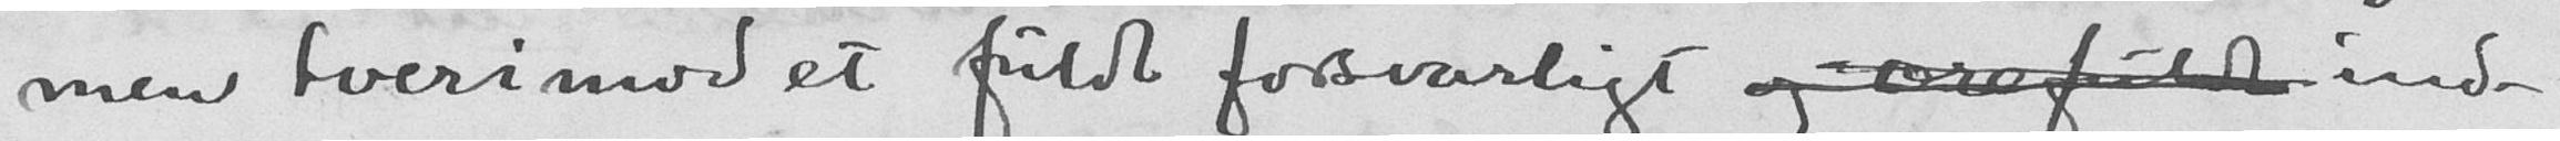men tvertimod et fuldt forsvarligt og ærefuldt ind-
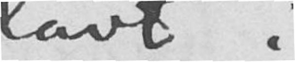lavt i
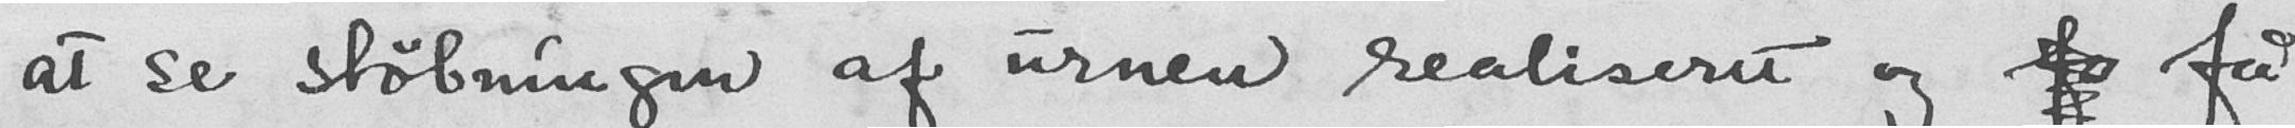at se støbningen af urnen realiseret og fa få
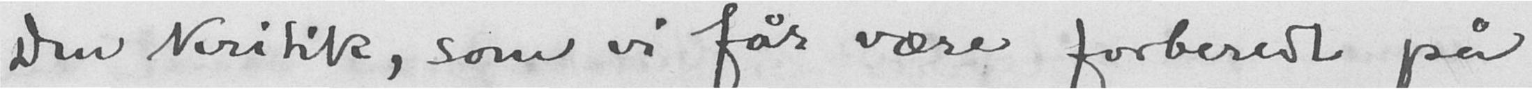Den kritik, som vi får være forberedt på
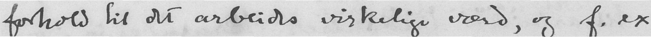forhold til det arbeids virkelige værd, og f.ex
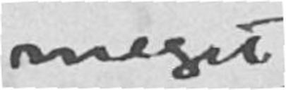meget
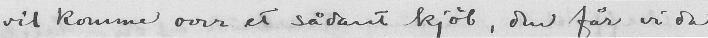vil komme over et sådant kjøb, den får vi da
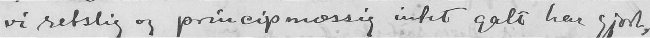vi retslig og principmæssig intet galt har gjort,
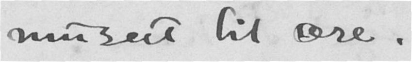museet til ære.
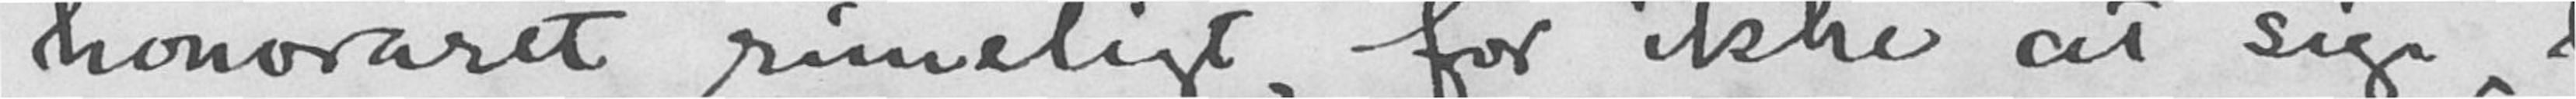honoraret rimeligt, for ikke at sige
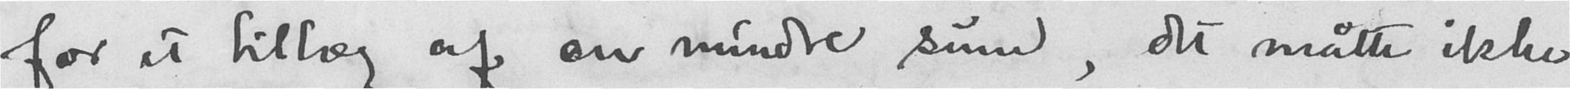for et tillæg af en mindre sum, det måtte ikke
